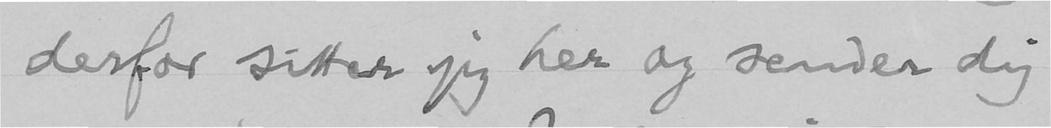derfor sitter jeg her og sender dig
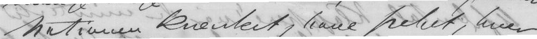Nationen Krenket, have pebet, hver
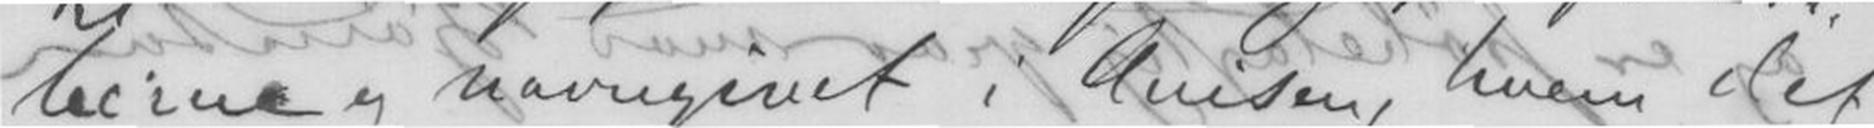tierne og navngivet i Avisen, hvem det
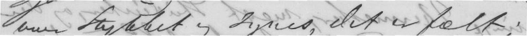over Stykket og synes, det er fælt;
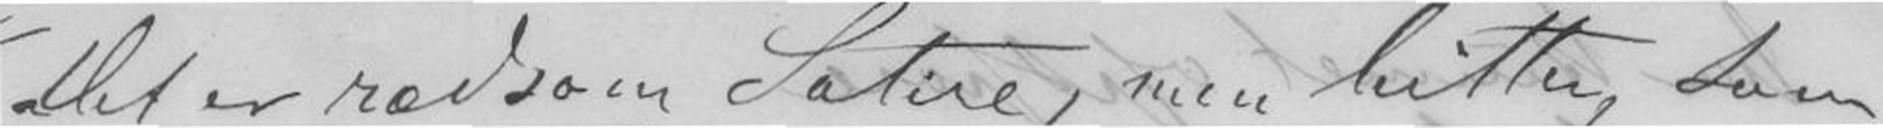Det er rædsom Satire, men lutter, som
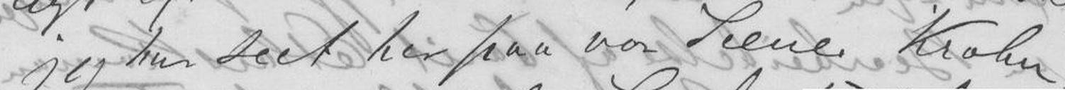jeg har seet her paa vor Scene. Krohn,
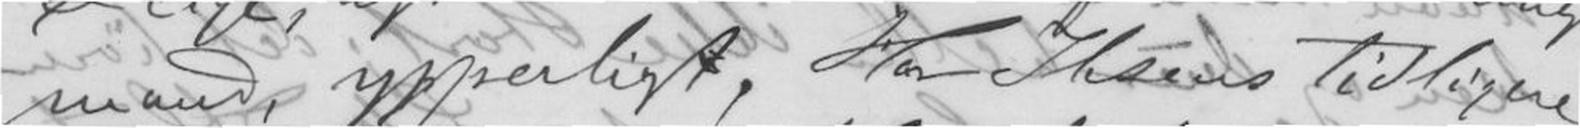mand, ypperligt. Har Ibsens Tidligere
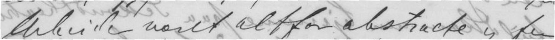Arbeide været altfor abstracte og for
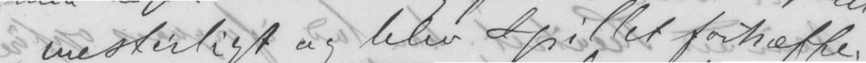mesterligt og blev Spillet fortræffe-
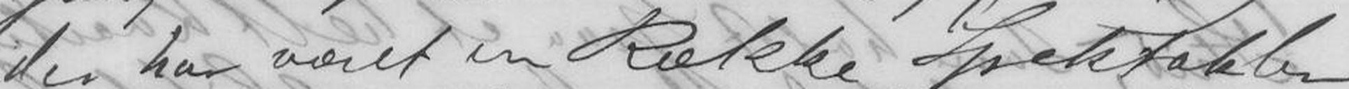der har været en Række Spetakler
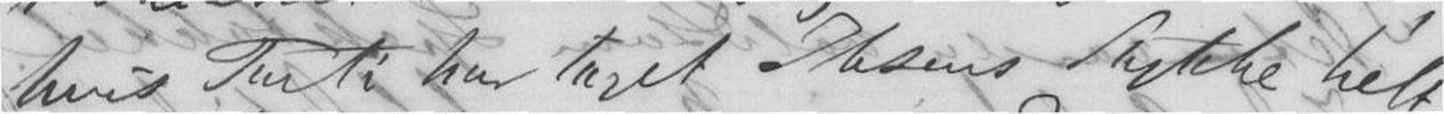hvis Parti har taget Ibsens Stykke helt
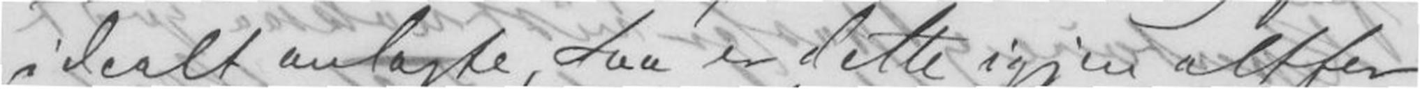idealt anlagt, saa er dette igjen altfor
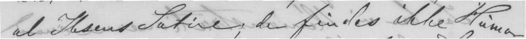al Ibsens Satire; der findes ikke Humør,
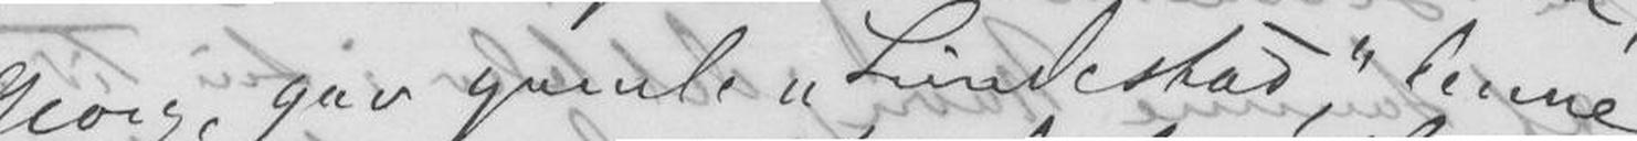Georg, gav geniale Lundstad, denne
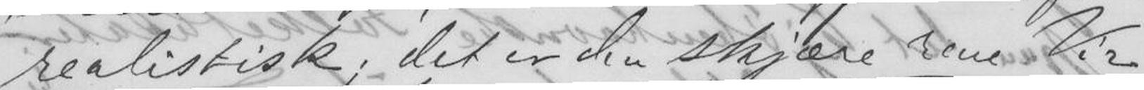realistisk; det er den skjære rene Vir-
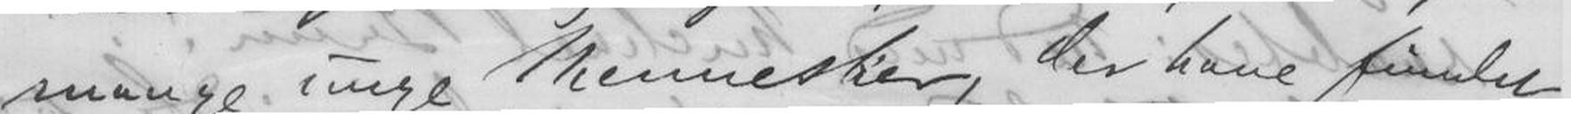mange unge Mennesker, der have fundet
